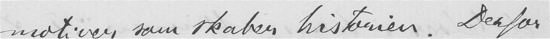motiver, som skaber historien. Derfor
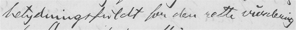betydningsfuldt for den rette vurdering
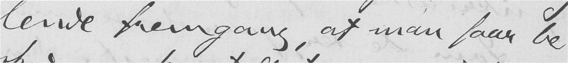lende fremgang, at man faar be-
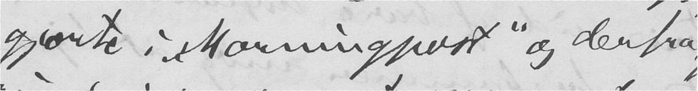gjorte i "Morningpost" og derfra
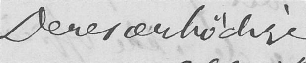Deres ærbødige
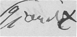gjort
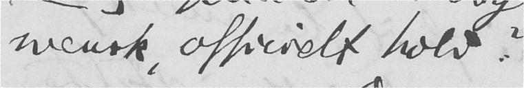svensk officielt hold?
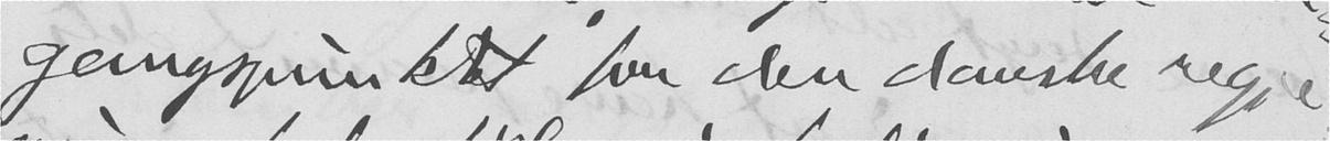gangspunktet for den danske regje-
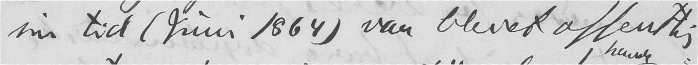sin tid (Juni 1864) var blevet offentlig-
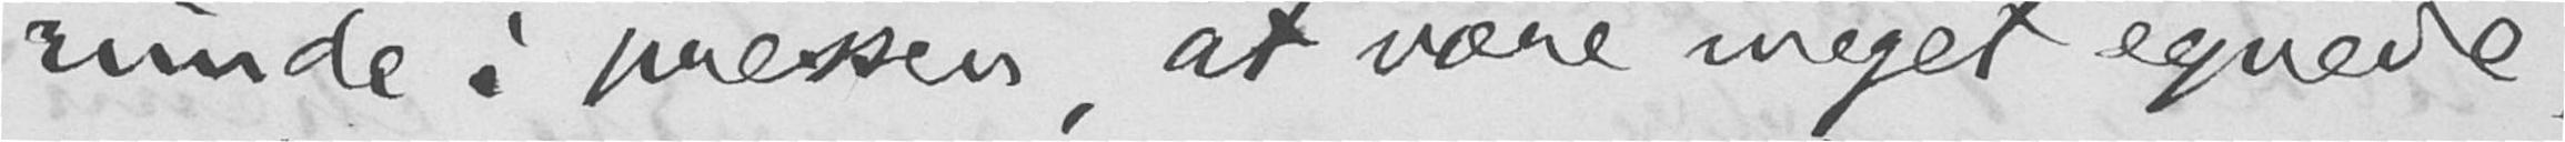runde i pressen, at være meget egnede.
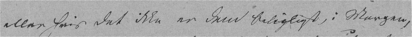eller hvis det ikke er Dem beleiligt, i Morgen,
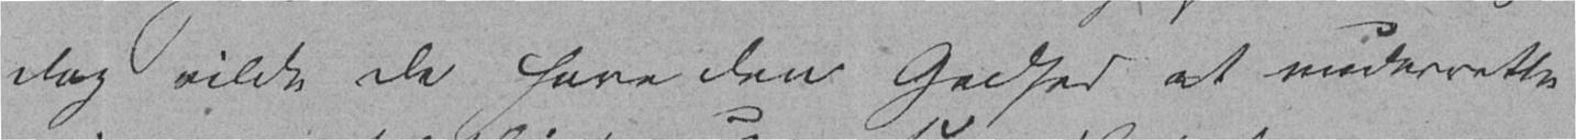dog vilde De have den Godhed at underrette
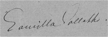Camilla Collett.
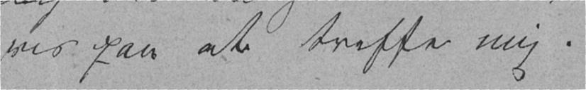vis paa at treffe mig.
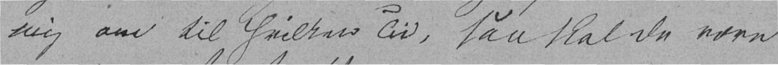mig om til hvilken Tid, saa skal De være
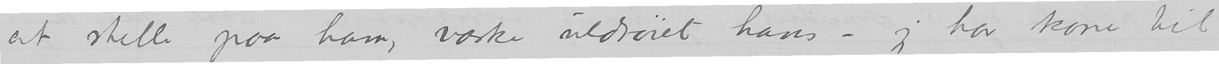at stelle paa ham, vaske uldtøiet hans – jeg har kone til
